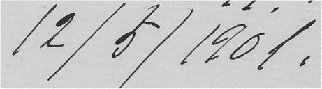12/5/1901.
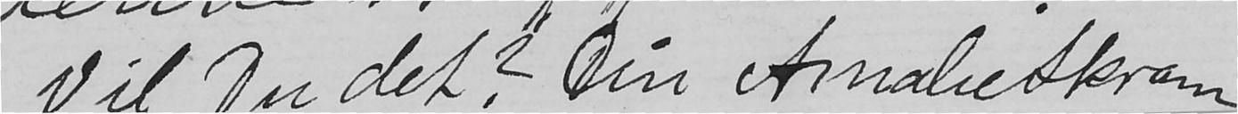Vil Du det? Din Amalie Skram
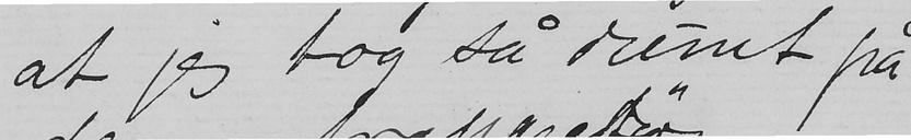at jeg tog så dumt på
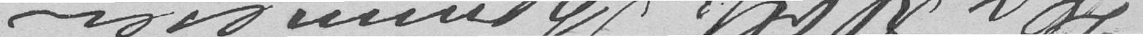Hr Rolf Thommesen
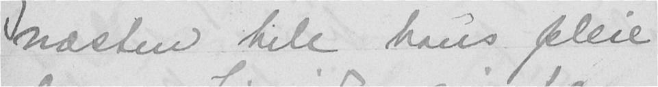næsten hele hans pleie
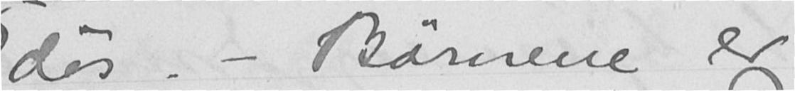døs. - Børnene er
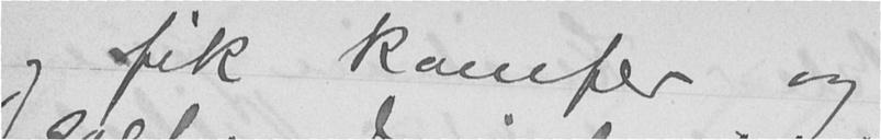og fik kamfer og
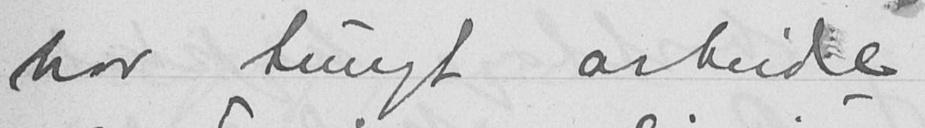har tungt arbeide
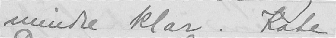mindre klar. Kate
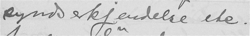syndserkjendelse etc.
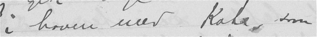i haven med Kate, som
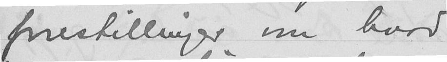forestillinger om hvad
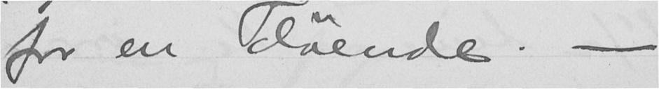for en døende. -
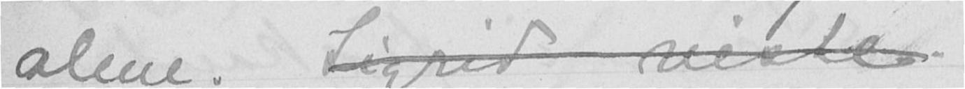alene. Sigrid viste
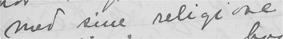med sine religiøse
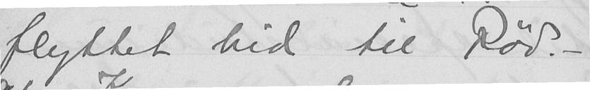flyttet hid til Rød. -
